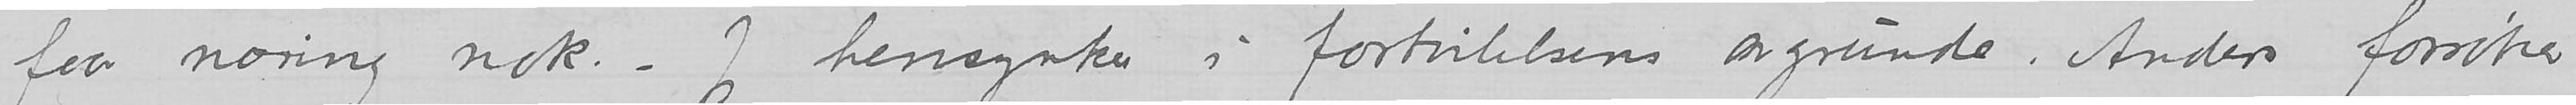faar næring nok. – Jeg hensynker i fortvilelsens avgrunde. Anders forsøker
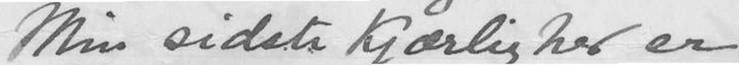Min sidste kjærlighed er
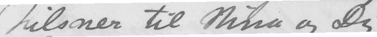hilsner til Nina og Dig
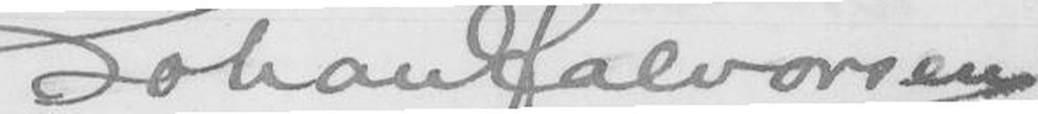Johan Halvorsen.
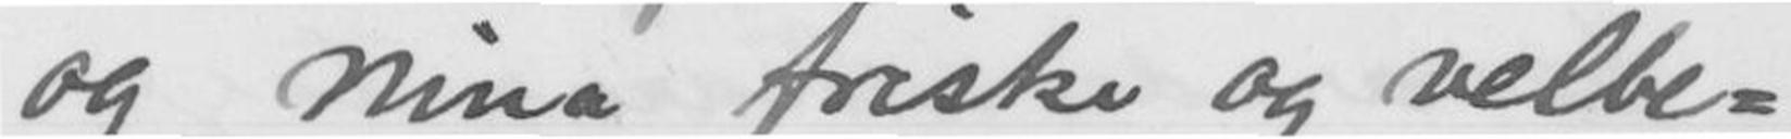og Nina friske og velbe-
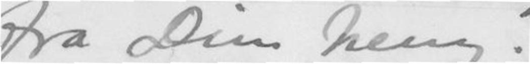fra Din heng.
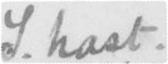I hast.
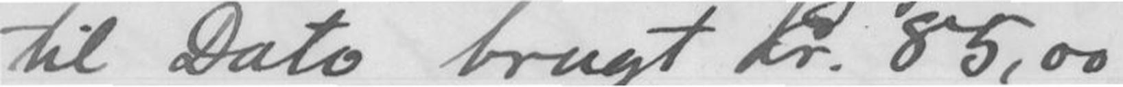til Dato brugt kr. 85,00
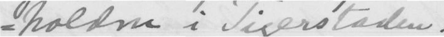holdne i Tigerstaden.
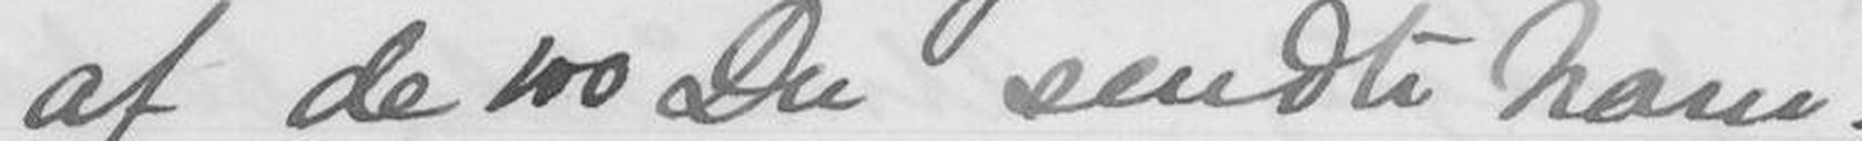af de 100 Du sendte ham.
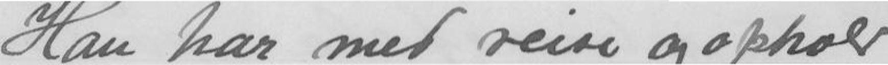Han har med reise og ophold
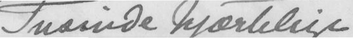Tusinde hjærtlige
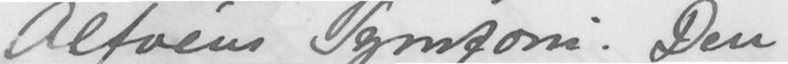Alfvéns Symfoni. Den
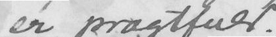er pragtfuld.
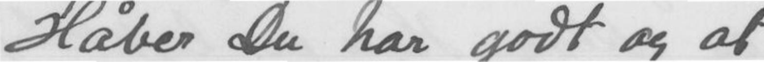Håber Du har godt og at
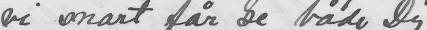vi snart får se både Dig
